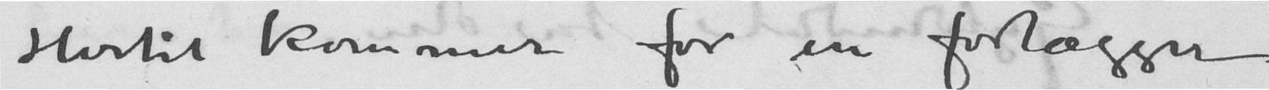Hertil kommer for en forlægger
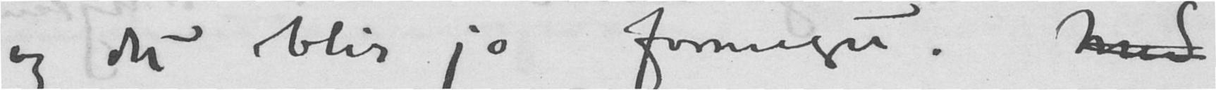og det blir jo formeget. Med
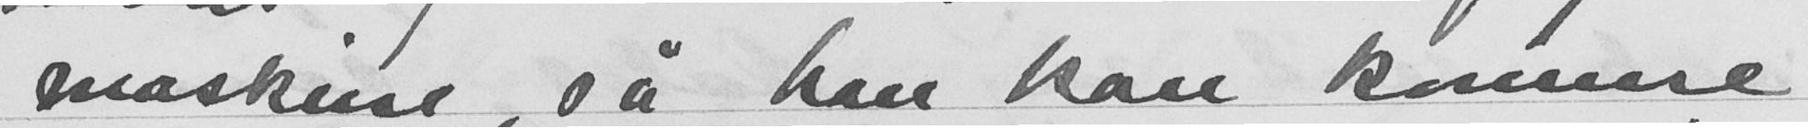maskin, så han kan komme
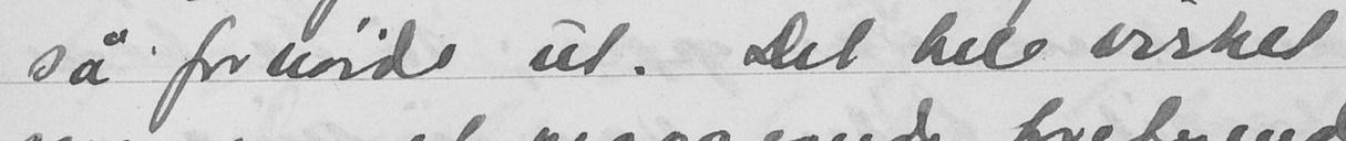så fornøide ut. Det hele virket
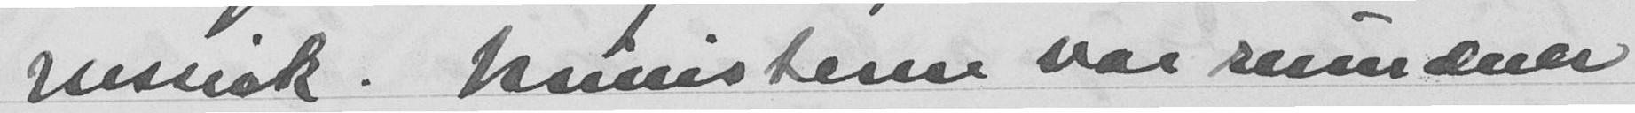russisk. Ministeren var rumener
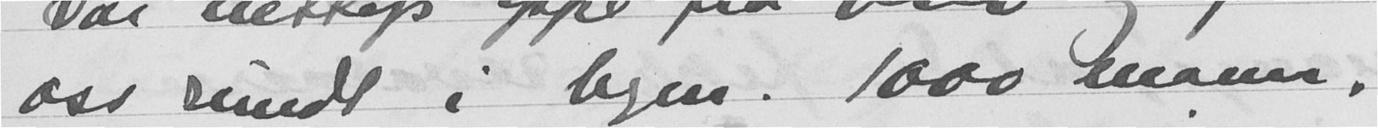oss rundt i byen. 1000 mann,
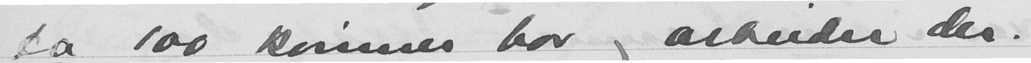ca 100 kvinner bor og arbeider der.
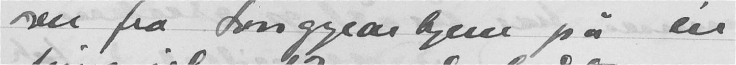over fra Longyearbyen på én
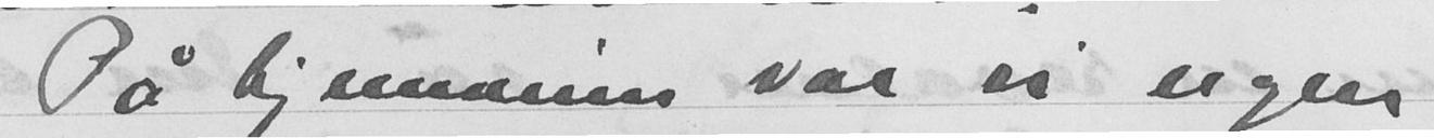På hjemveien var vi nogen
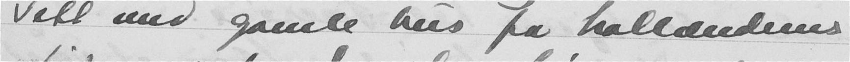Vell med gamle hus fra Hollændernes
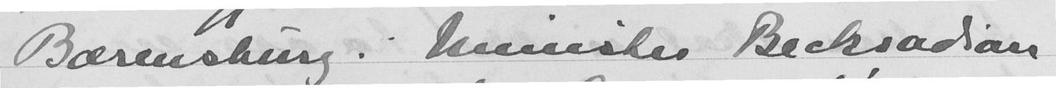Bærensburg. Minister Becksadian
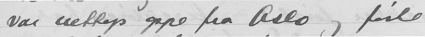var nettop oppe fra Oslo og førte
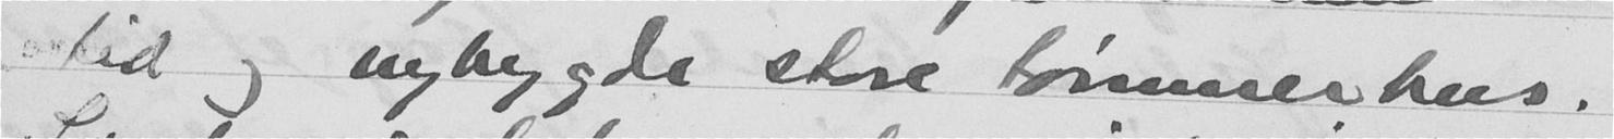tid og nybygde store tømmerhus.
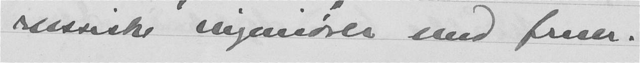russiske ingeniører med fruer.
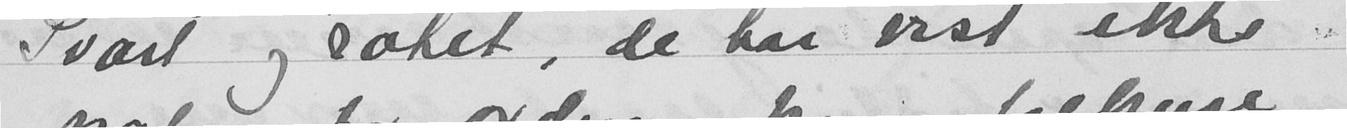Svart og sotet, de har vist ikke
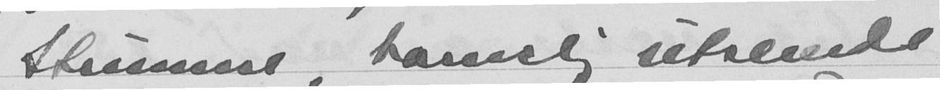Stumme, barnlig utseende
